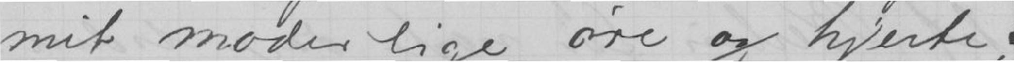mit moderlige øre og hjerte;
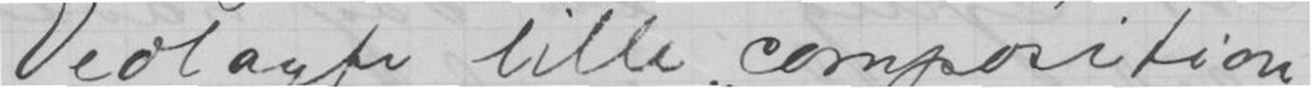Vedlagte lille composition
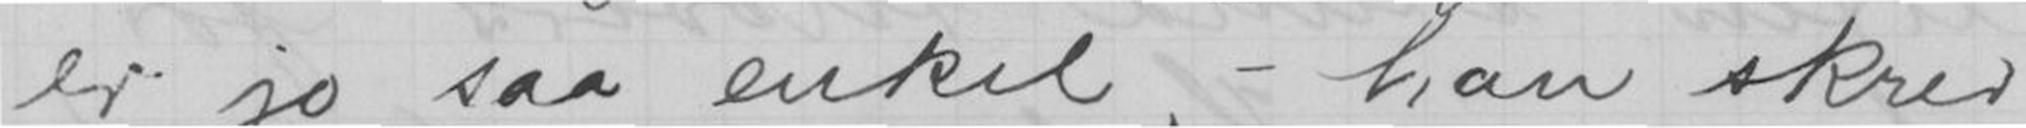er jo saa enkel,- han skrev
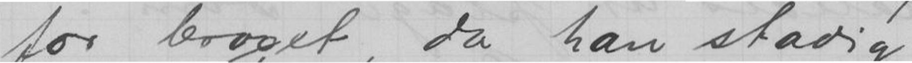for broget, da han stadig
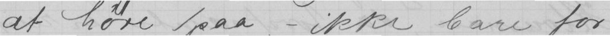at høre /paa,- ikke bare for
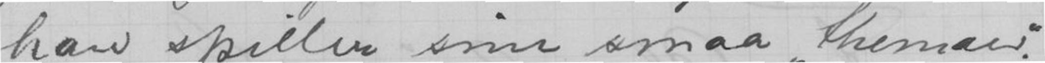han spiller sine smaa themaer
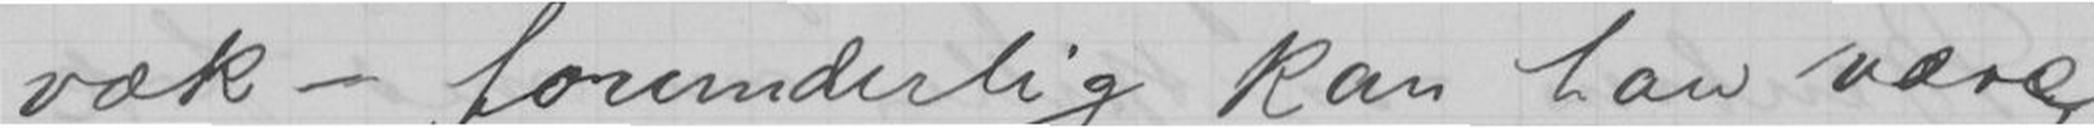væk- forunderlig kan han være
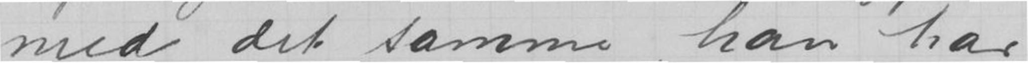med det samme, han har
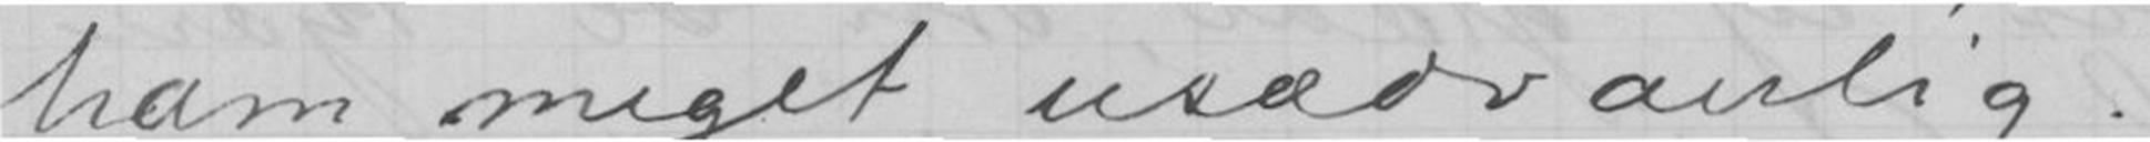ham meget usedvanlig.
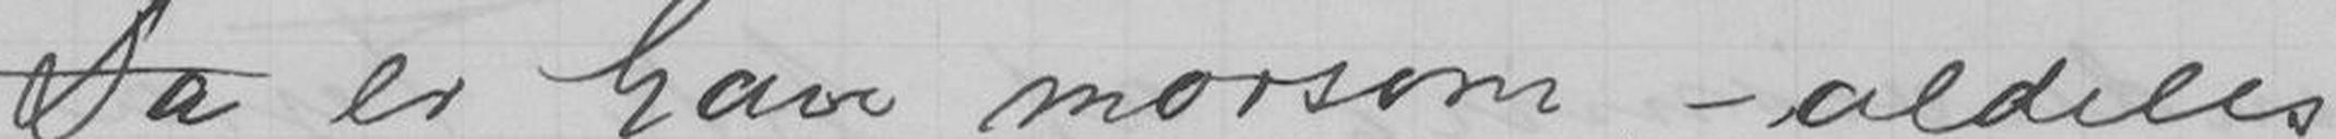Da er han morsom,- aldeles
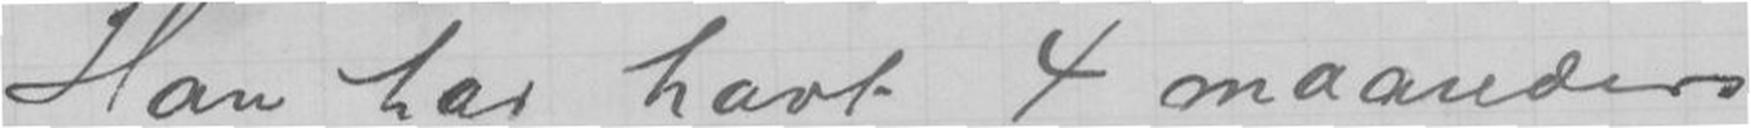Han har havt 4 maaneders
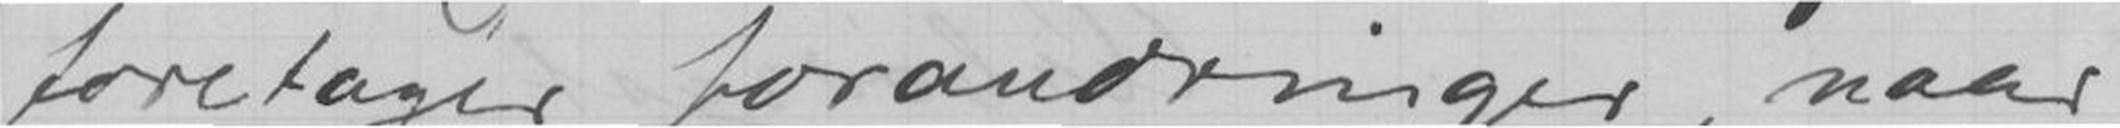foretager forandringer, naar
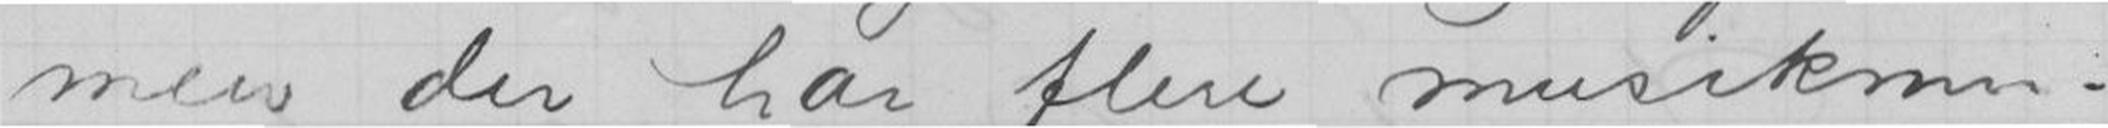men der har flere musikmen-
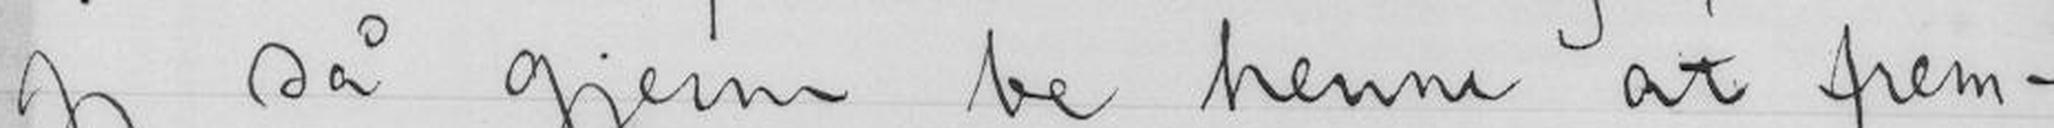jeg så gjerne be henne at frem-
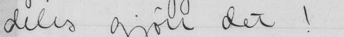deles gjøre det!
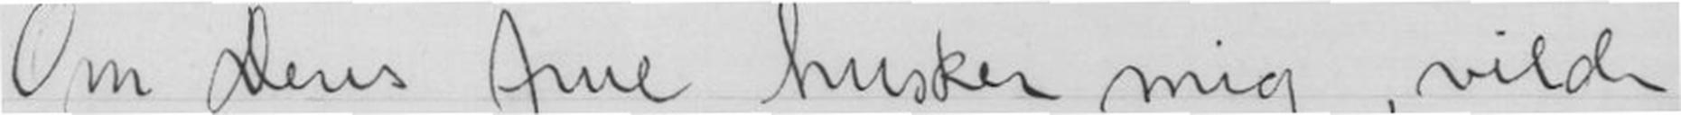Om Deres frue husker mig, vilde
